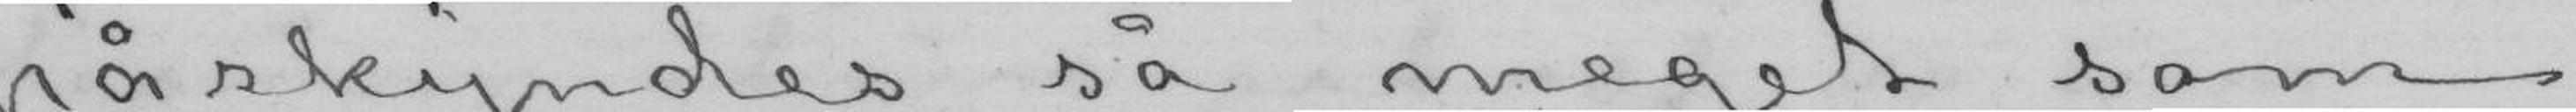påskyndes så meget som
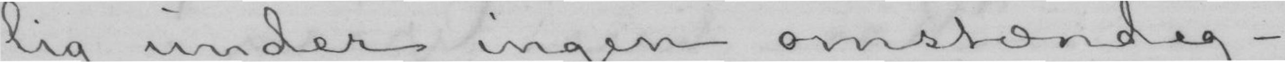lig under ingen omstændig-
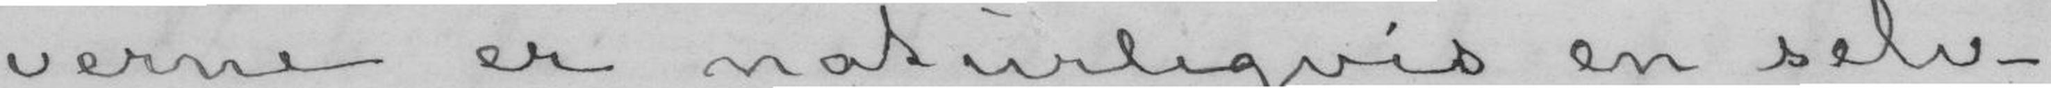verne er naturligvis en selv-
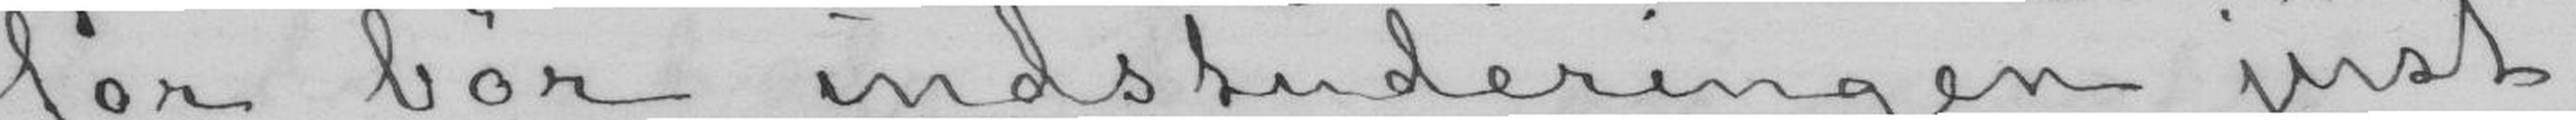for bør indstuderingen just
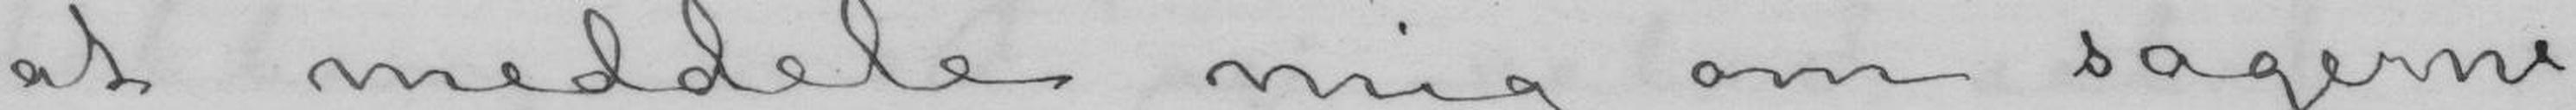at meddele mig om sagerne,
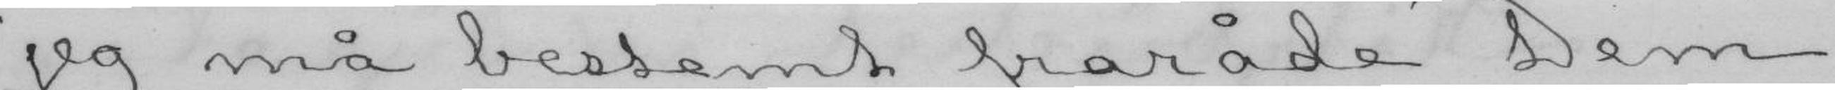jeg må bestemt fraråde Dem
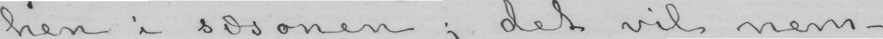hen i sæsonen; det vil nem-
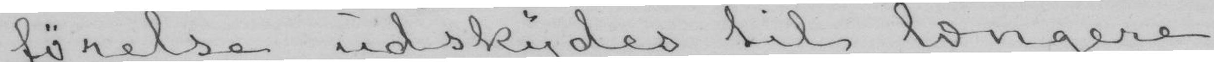førelse udskydes til længere
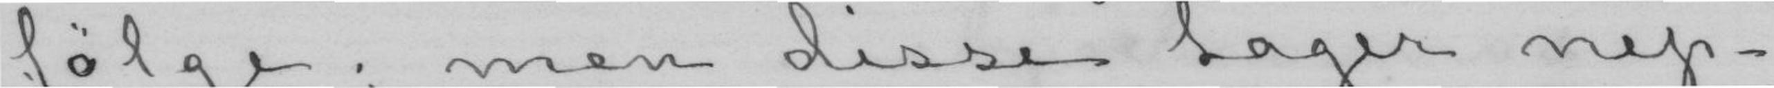følge; men disse tager nep-
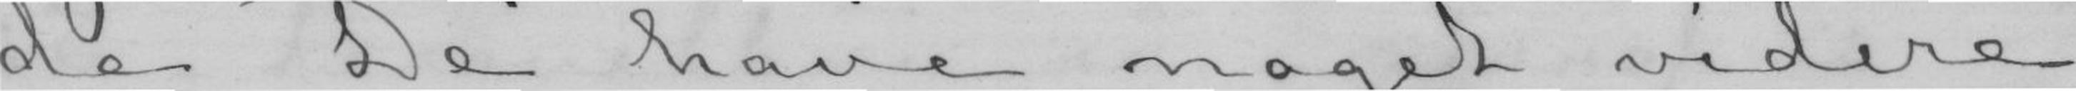de De have noget videre
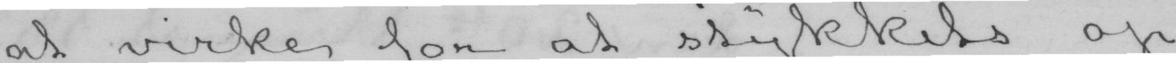at virke for at stykkets op
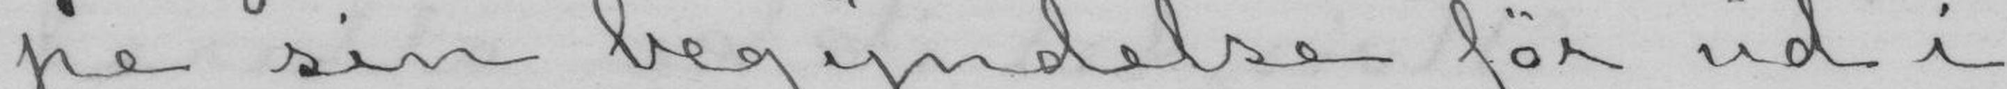pe sin begyndelse før ud i
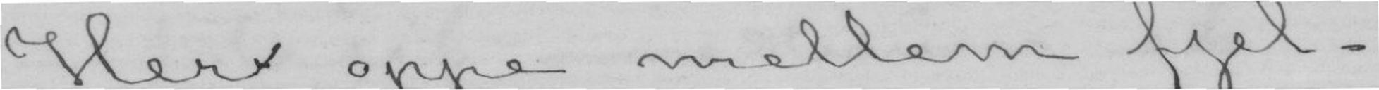Her oppe mellem fjel-
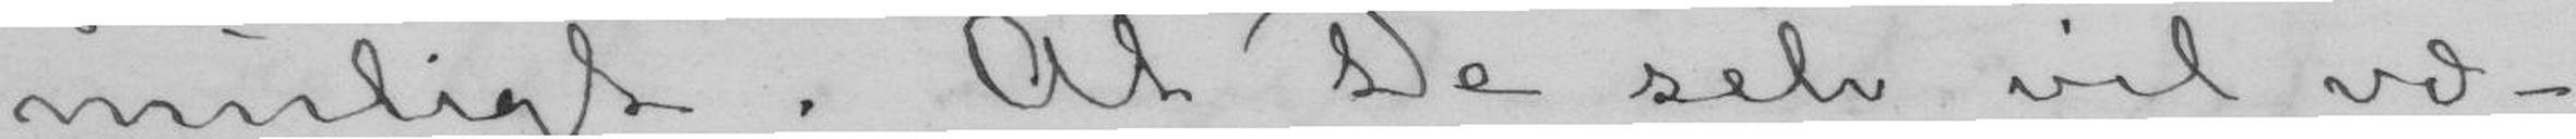muligt. At De selv vil væ-
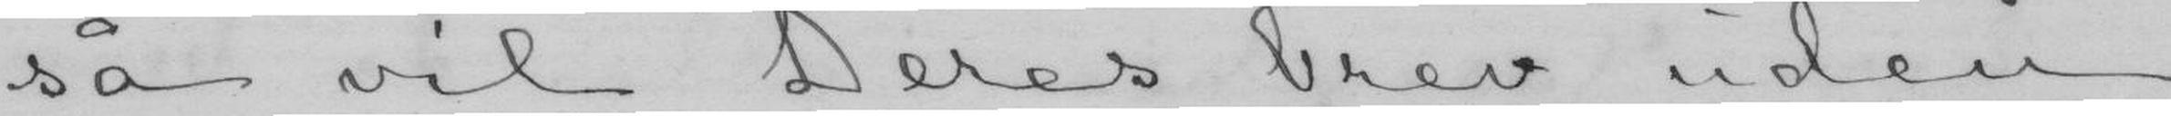så vil Deres brev uden
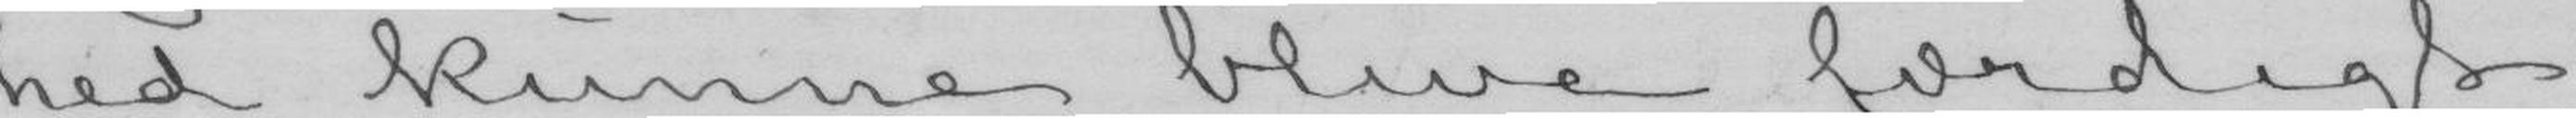hed kunne blive færdigt
